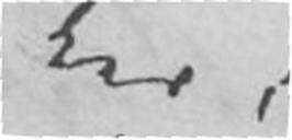ker,
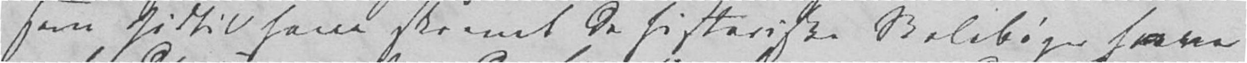som hidtil have skrevet de historiske Skolebøger have
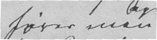føres man
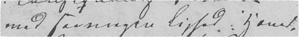med saamegen Lighed: Hoved-
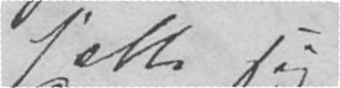sætte sig
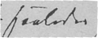saaledes
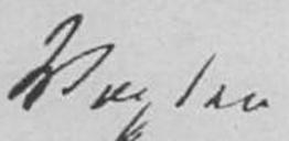Væxten
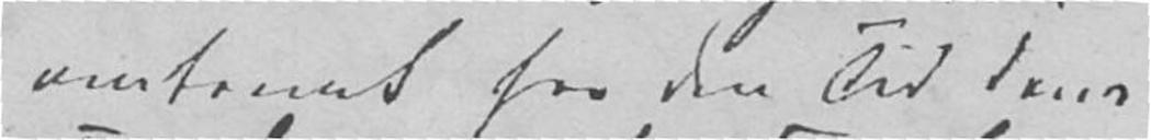omtrent fra den Tid dens
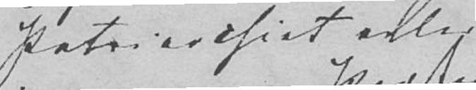Patriarchiet eller
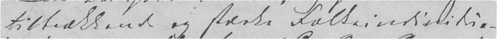tiltrækkende og stærke Folkeindustria-
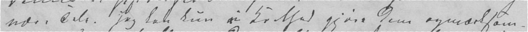være Tale. Jeg kan kun i Korthed gjøre Dem opmærksom-
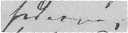hederne,
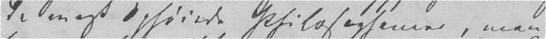de mest ophøiede Philosopherne, men
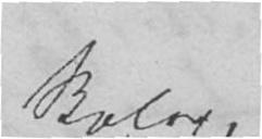Skoler,
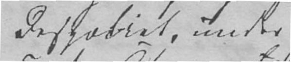Despotiet, under
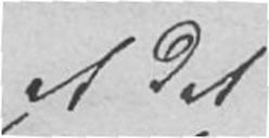at det
